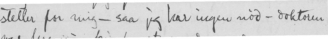steller for mig - saa jeg har ingen nød - doktoren
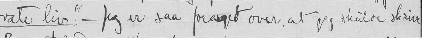vate liv". - Jeg er saa forarget over, at jeg skulde skriv
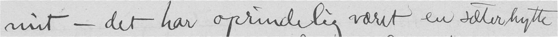mit - det har oprindelig været en sæterhytte
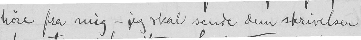høre fra mig - jeg skal sende dem skrivelsen
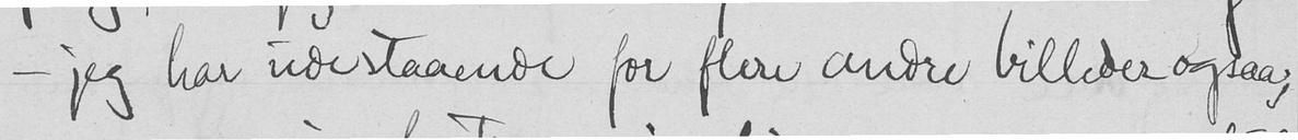- jeg har udestaaende for flere andre billeder ogsaa;
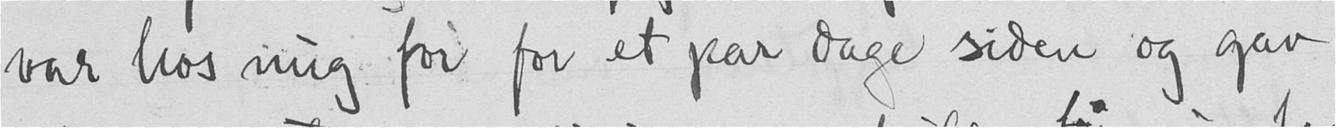var hos mig for for et par dage siden og gav
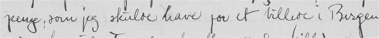penge, som jeg skulde have for et billede i Bergen
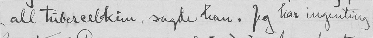all tubercel-kim, sagde han. Jeg har ingenting
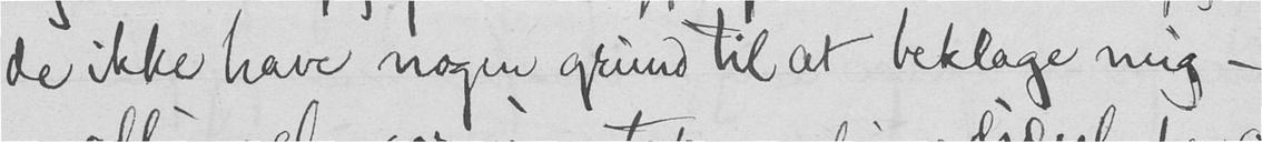de ikke have nogen grund til at beklage mig -
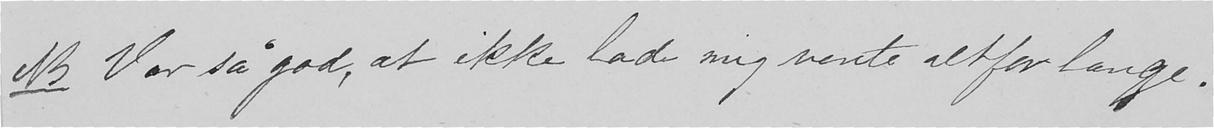NB Vær så god, at ikke lade mig vente altfor længe.
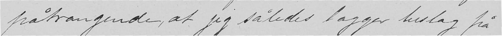påtrængende, at jeg således lægger beslag på
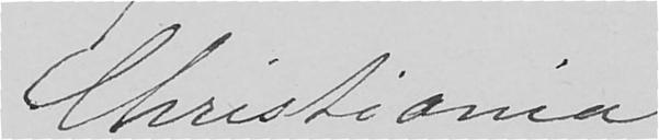Christiania
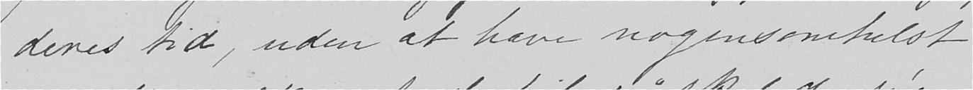deres tid, uden at have nogensomhelst
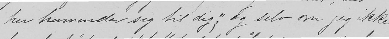her henvender sig til dig;" og selv om jeg ikke
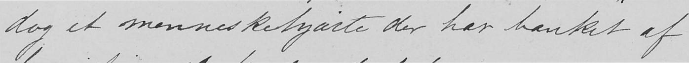dog et menneskehjærte der har banket af
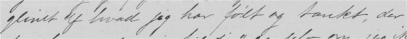glimt af hvad jeg har følt og tankt, der
